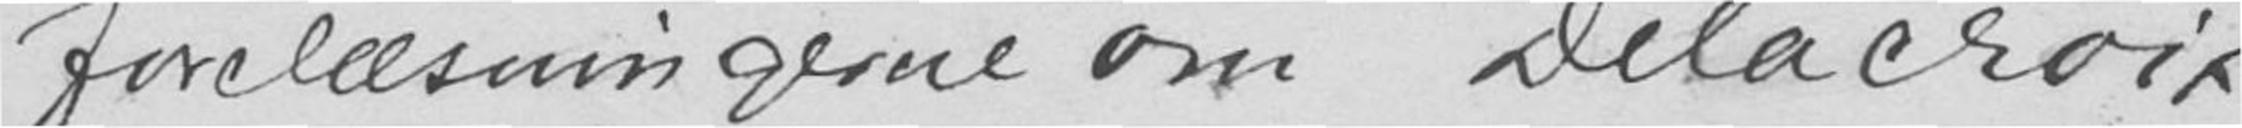forelæsningene om Delacroix
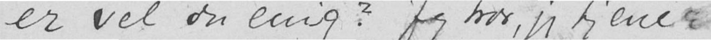er vel du enig? Jeg tror, jeg tjener
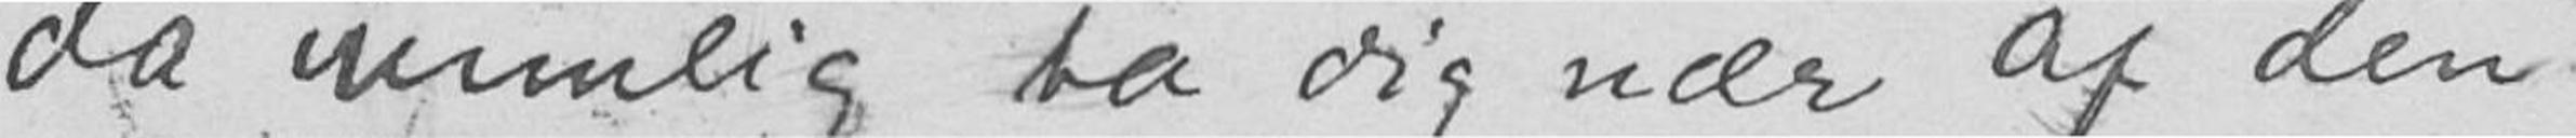da umulig ta dig nær af den
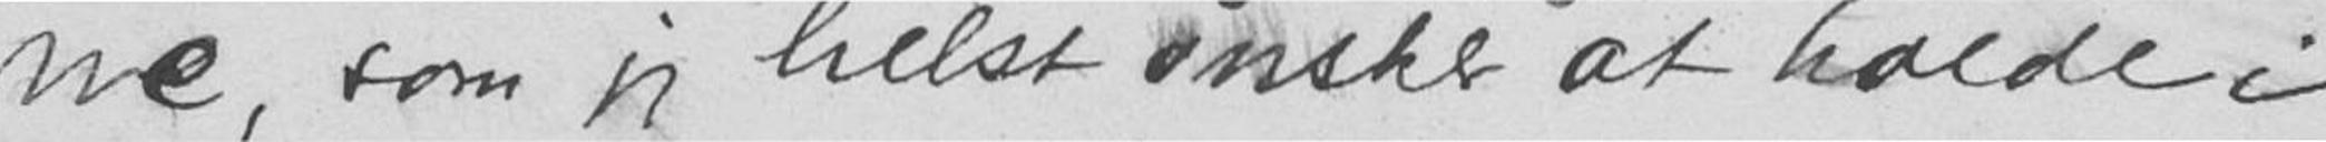ne, som jeg helst ønsker at holde i
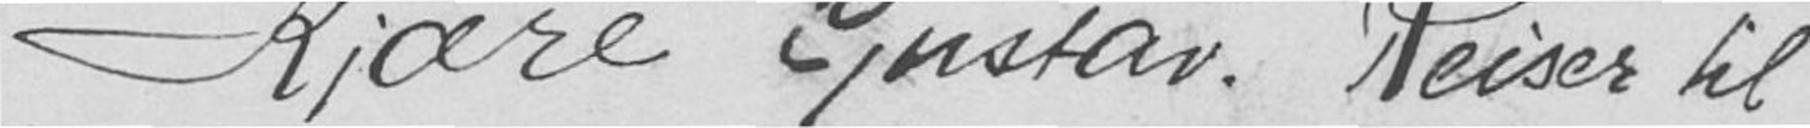Kjære Gustav. Reiser til
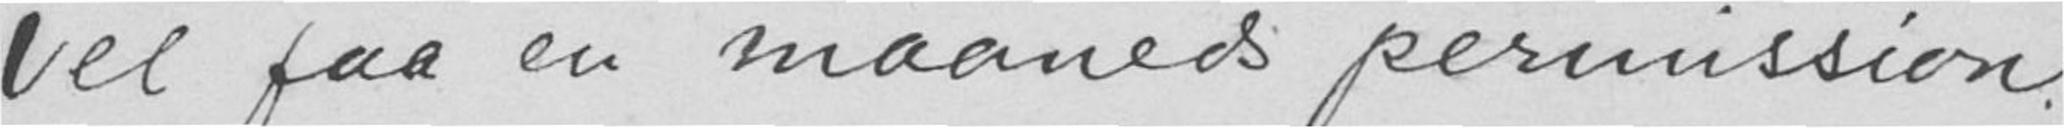vel faa en maaneds permission.
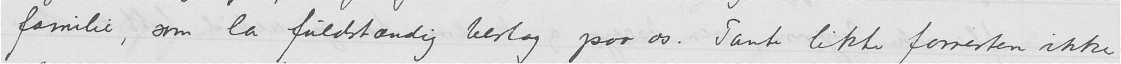familie, som la fuldstændig beslag paa os. Tante likte forresten ikke
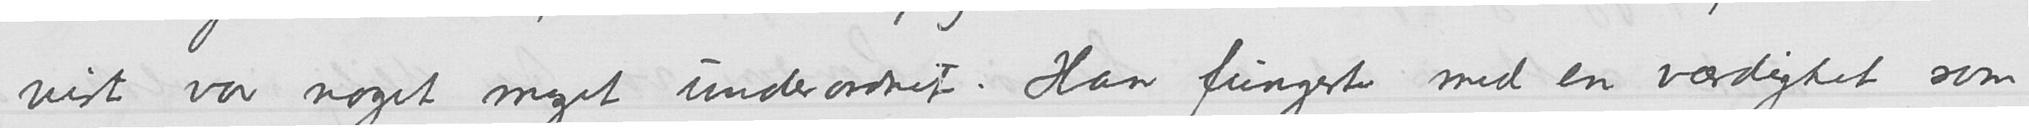vist var noget meget underordnet. Han fungerte med en værdighet som
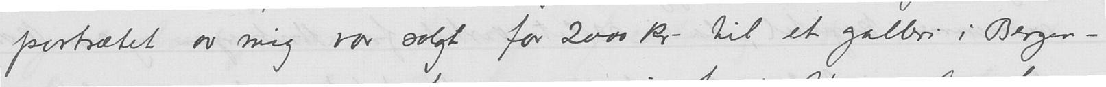portrætet av mig var solgt for 2000 kr. til et galleri i Bergen -
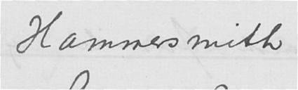Hammersmith
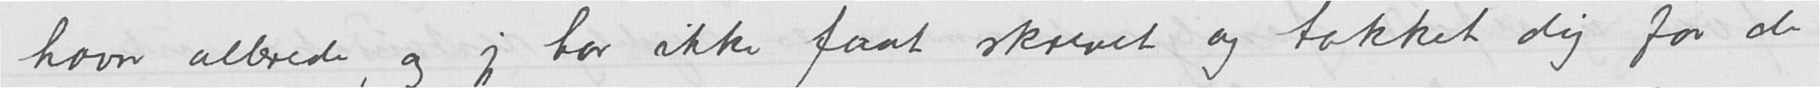havn allerede, og jeg har ikke faat skrevet og takket dig for de
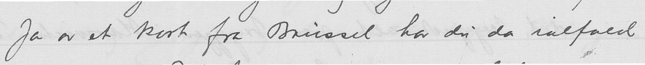Ja av et kort fra Brussel har du da ialfald
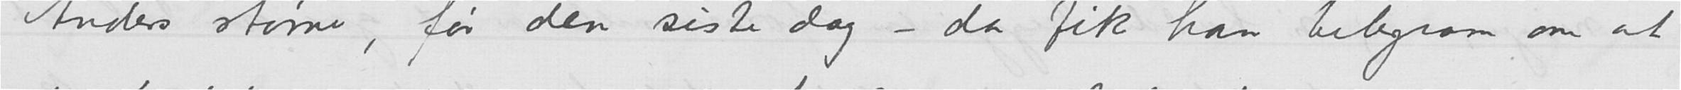Anders større, før den siste dag — da fik han telegram om at
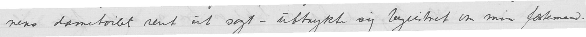nens dametoilet rent ut sagt - uttrykte sig begeistret om min fæstemand.
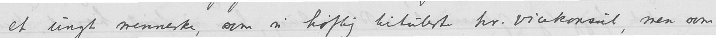et ungt menneske, som vi høflig titulerte hr. vicekonsul, men som
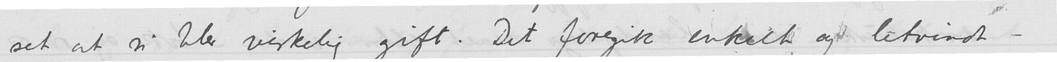set at vi blev virkelig gift. Det foregik enkelt og letvindt -
